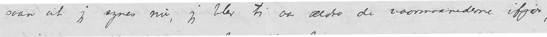saan at jeg synes nu, jeg blev ti aar ældre de vaarmaanederne ifjor,
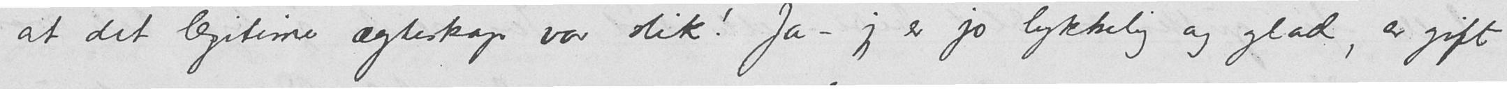at det legitime ægteskap var slik! Ja - jeg er jo lykkelig og glad, er gift
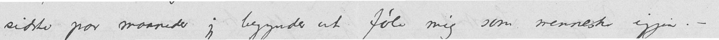sidste par maaneder jeg begynder at føle mig som menneske, igjen. -
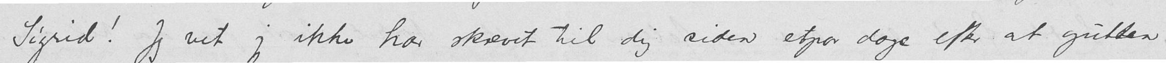Sigrid! Jeg vet jeg ikke har skrevet til dig siden etpar dage efter at gutten
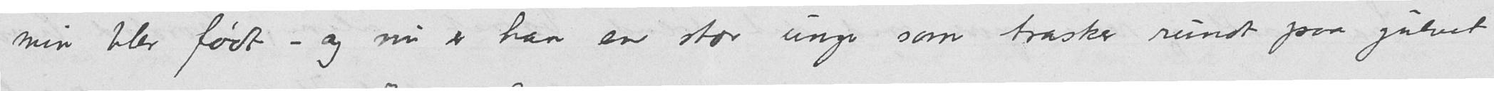min blev født - og nu er han en stor unge som trasker rundt paa gulvet
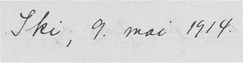Ski, 9. mai 1914.
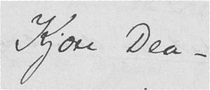Kjære Dea -
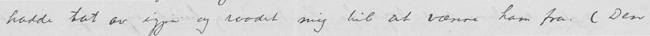hadde tat av igjen og raadet mig til at vænne ham fra. (Den
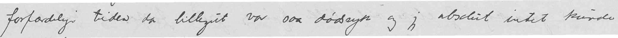forfærdelige tiden da lillegut var saa dødssyk og jeg absolut intet kunde
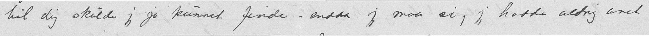til dig skulde jeg jo kunnet finde - endda jeg maa si, jeg jeg hadde aldrig anet,
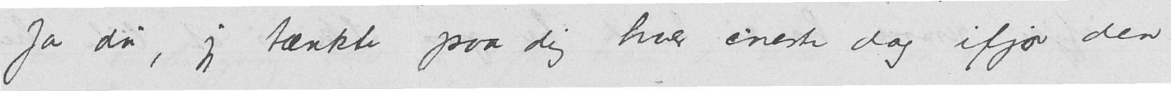Ja du, jeg tænkte paa dig hver eneste dag ifjor den
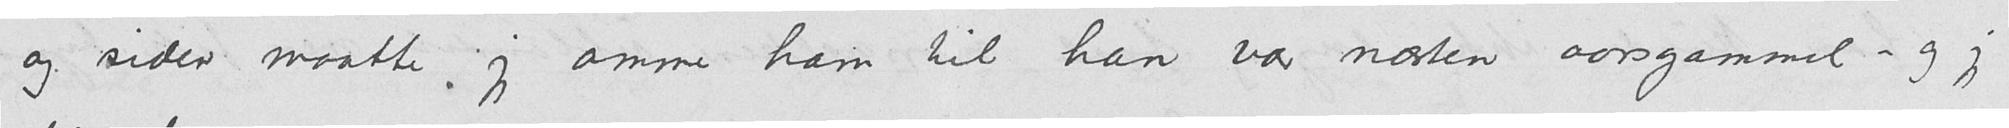og siden maatte jeg amme ham til han var næsten aarsgammel - og jeg
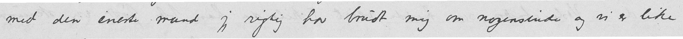med den eneste mand jeg rigtig har brudt mig om nogensinde og vi er like
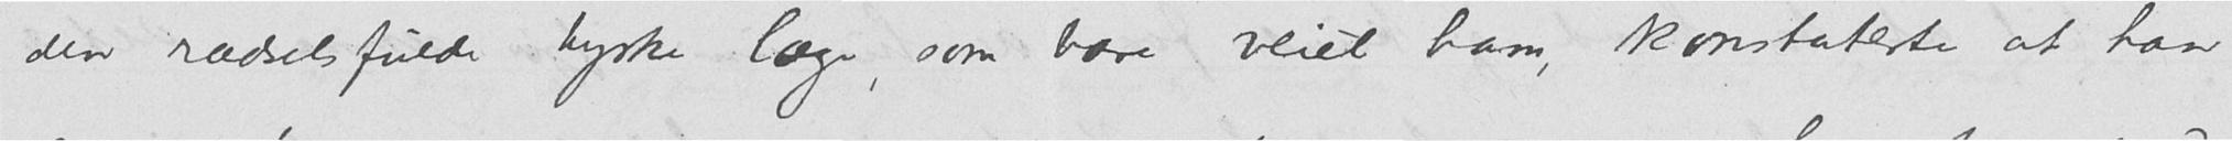den rædselsfulde tyske læge, som bare veiet ham, konstaterte at han
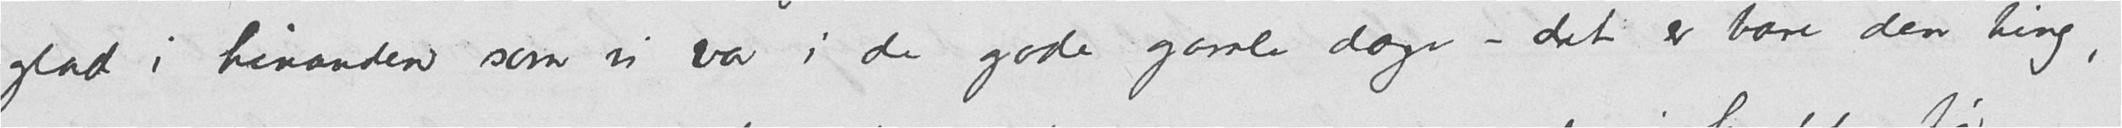glad i hinanden som vi var i de gode gamle dage - det er bare den ting,
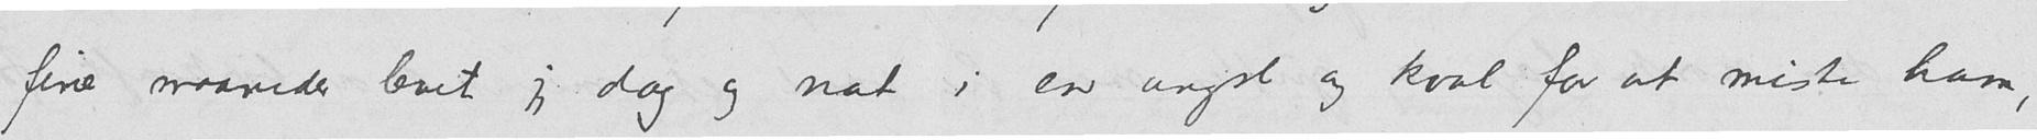fire maaneder levet jeg dag og nat i en angst og kval for at miste ham,
